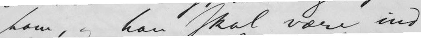ham, og han skal være ind-
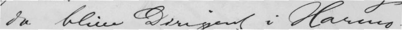da blive Dirigent i Harmo-
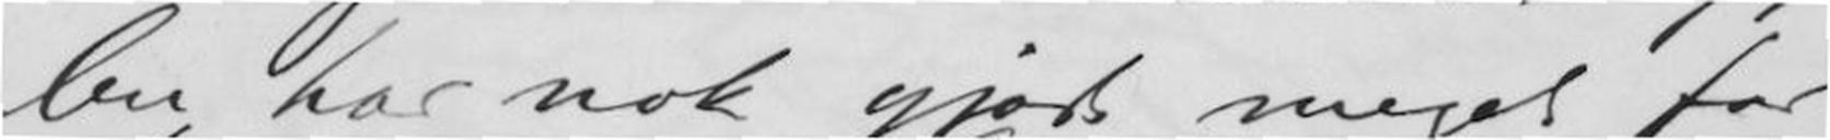len har nok gjort meget for
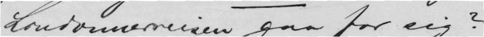Londonnerreisen gaa for sig?
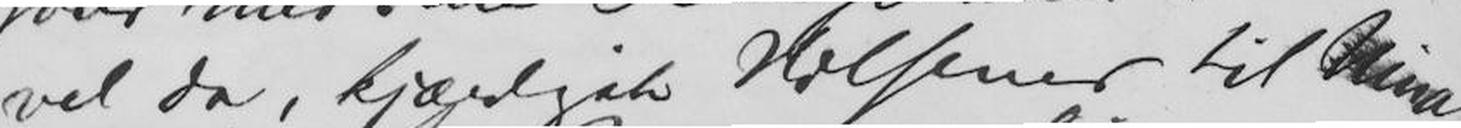vel da, kjærligste Hilsener til Nina
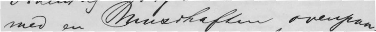med en Musikhaften ovenpaa,
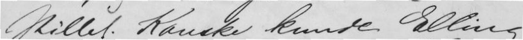stillet. Kanske kunde Elling
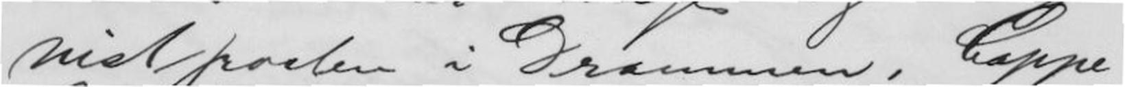nistposten i Drammen. Cappe-
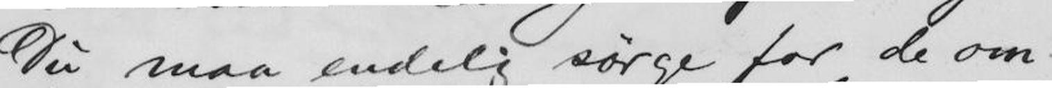Du maa endelig sørge for de om-
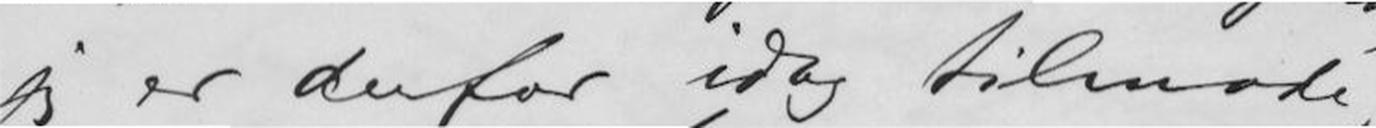og jeg er derfor idag tilmode,
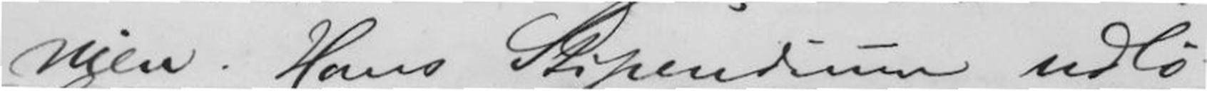nien. Hans Stipendium udlø-
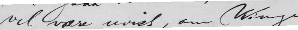vel være uvist, om Winge
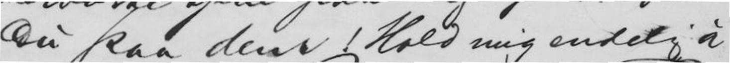Du paa dem! Hold mig endelig à
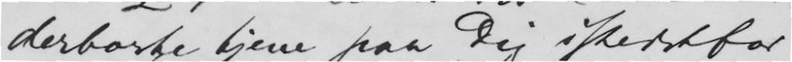derborte tjene paa Dig istedetfor
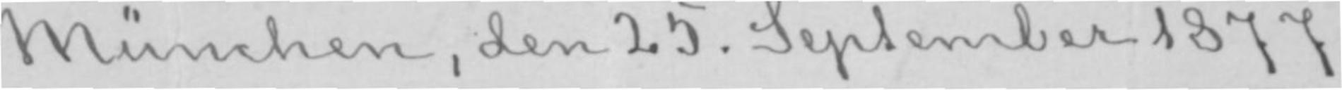München, den 25. September 1877.
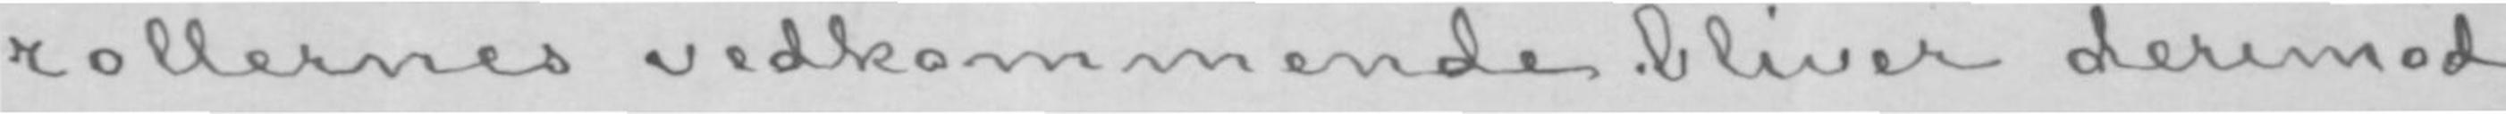rollernes vedkommende bliver derimod
